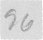96
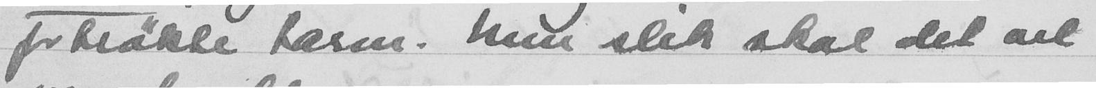forkrøkte tarm. Men slik skal det ant
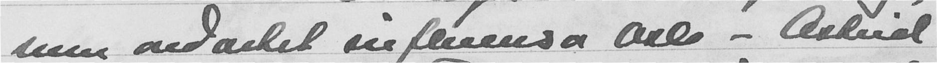som ondartet influensa Oslo - Astrid
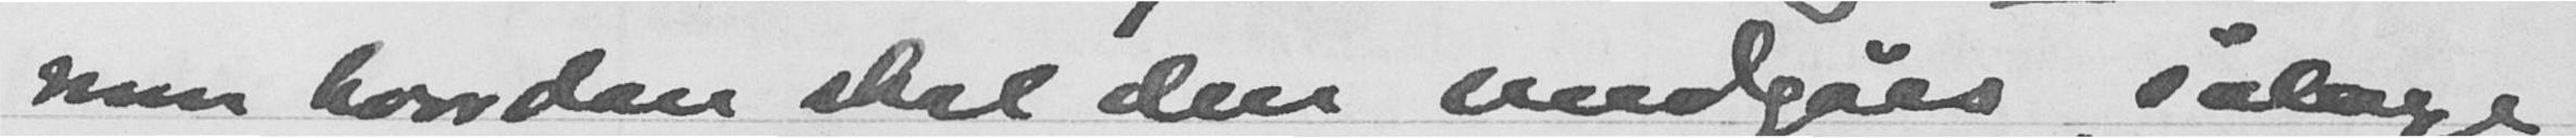men hvordan skal den undgåes, sålenge
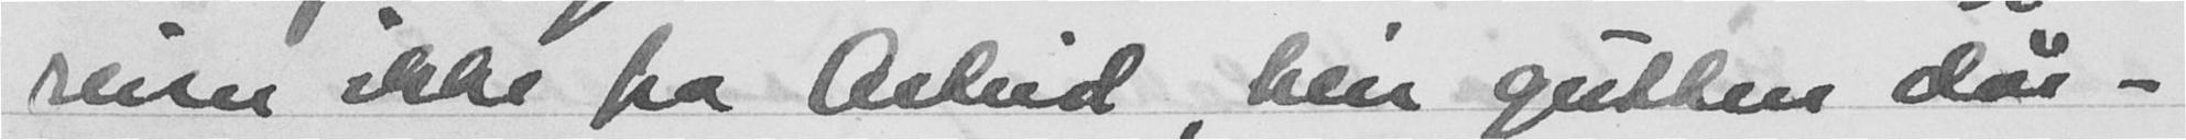reiser ikke fra Astrid, blir gutten dår-
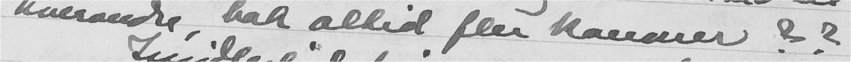hverandre, bak altid fler kanoner??
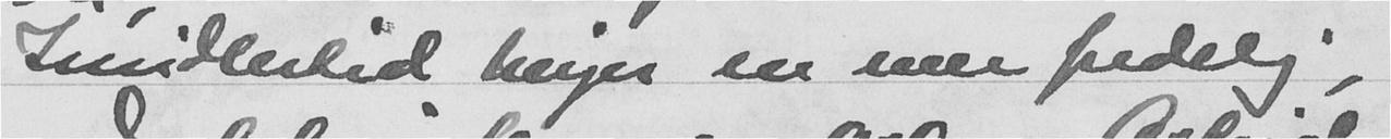Imidlertid herjer en ener fredelig,
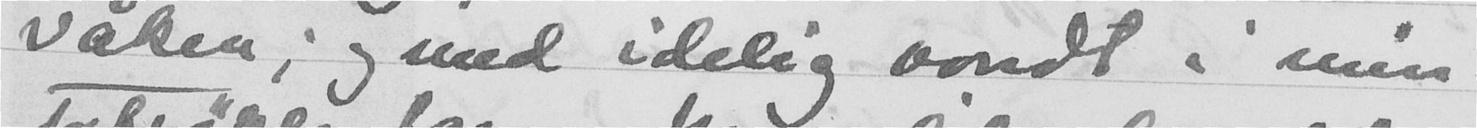våken; og med idelig vondt i min
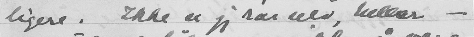ligere. Ikke er jeg sår selv, heller -
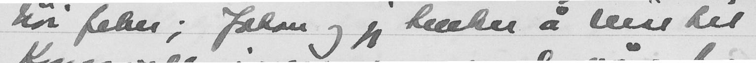høi feber; Johan og jeg tenker å reise til
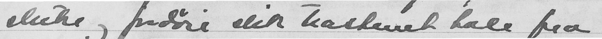sluke og fordøse slik hastenet tale fra
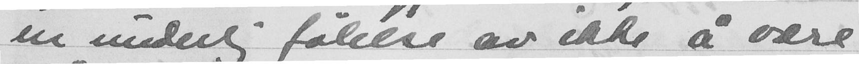en underlig følelse av ikke å være
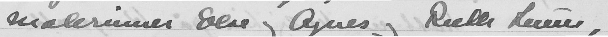malerinner Else og Agnes og Riette Leum,
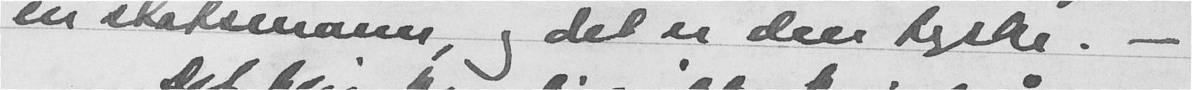en statsmann, og det er den tyske. -
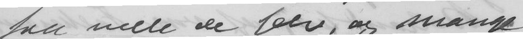saa vilde de selv, og mange
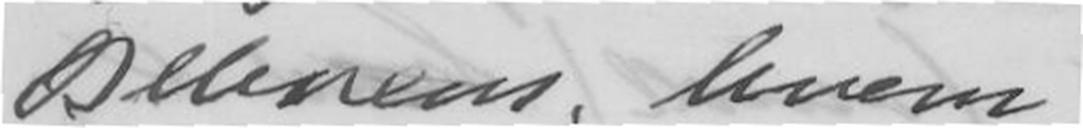Behrens, hvem
